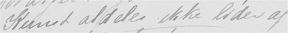Kunst aldeles ikke lider af
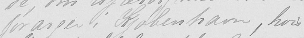forarger i Kjøbenhavn, hvis
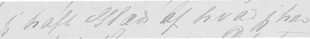jeg haft Glæde af hvad jeg har
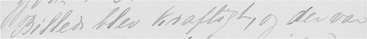Billede blev kraftigt, og der var
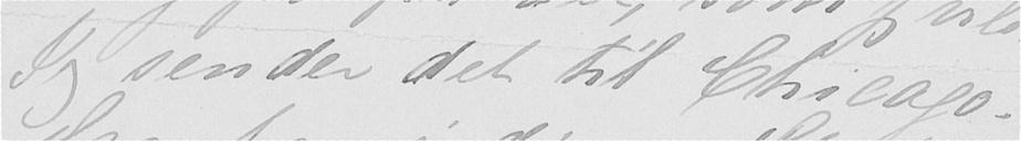Jeg sender det til Chigago.
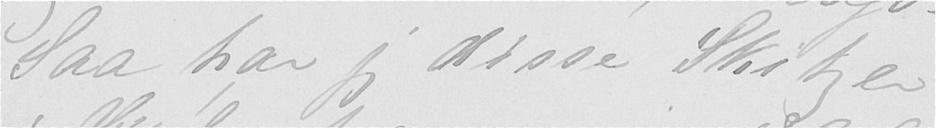Saa har jeg disse Skitzer
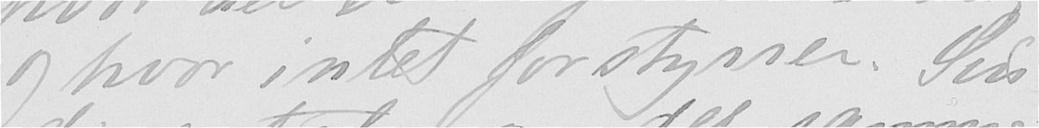og hvor intet forstyrrer. Sin-
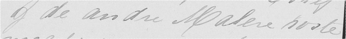og de andre Malere roste
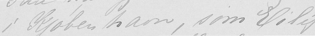i Kjøbenhavn, som Eilif
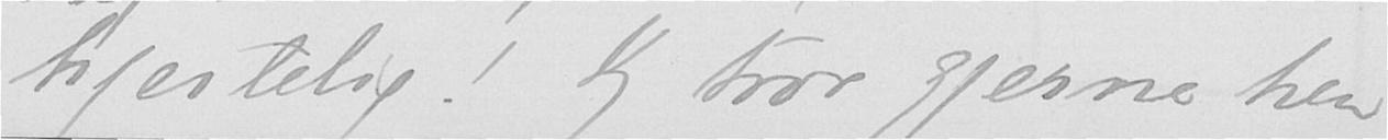hjertelig! Jeg tror gjerne hen-
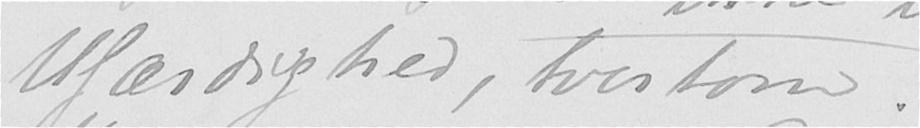Ufærdighed, tvertom.
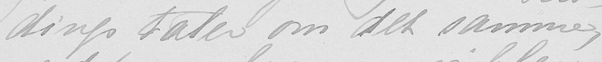dings taler om det samme,
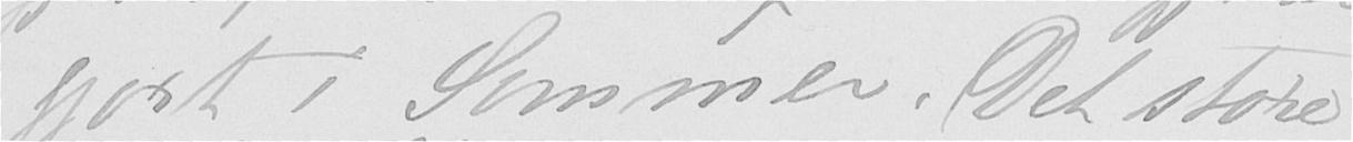gjort i Sommer. Det store
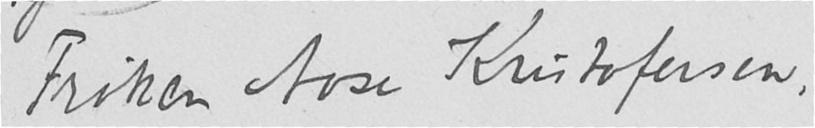Frøken Aase Kristofersen,
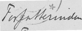Forfatterinden
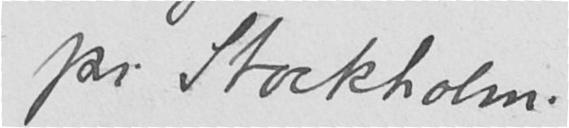pr. Stockholm.
